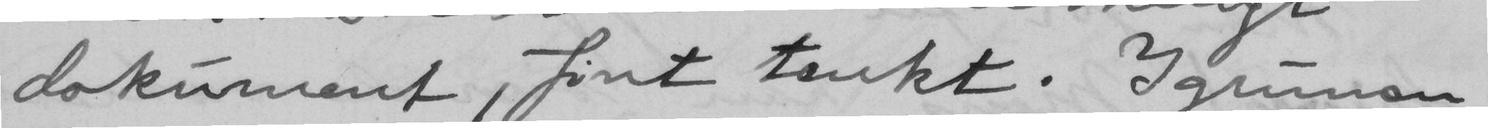dokument, fint tenkt. Igrunnen
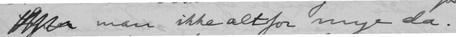man ikke alt for mye da.
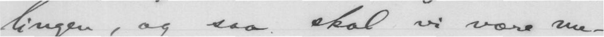lingen, og saa skal vi være me-
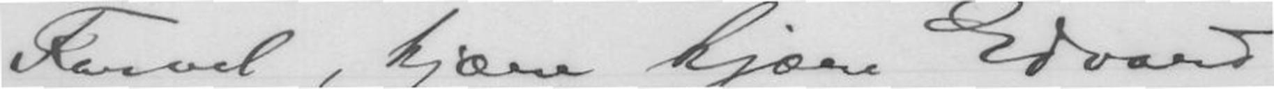Farvel, kjære kjære Edvard!
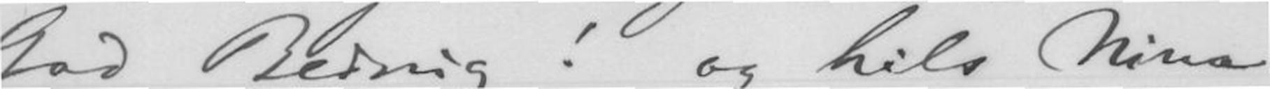God Bedring! og hils Nina
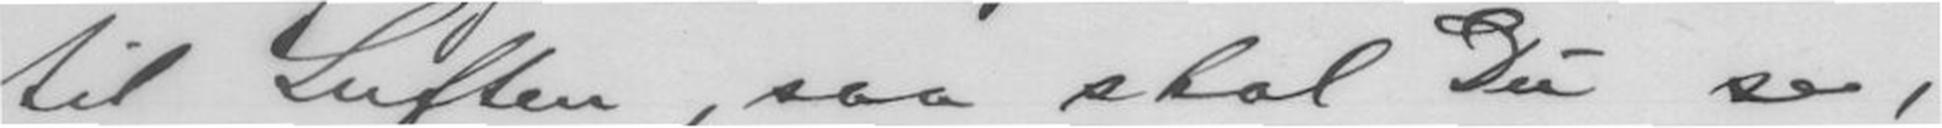til Luften, saa skal Du se,
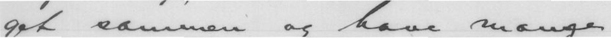get sammen og have mange
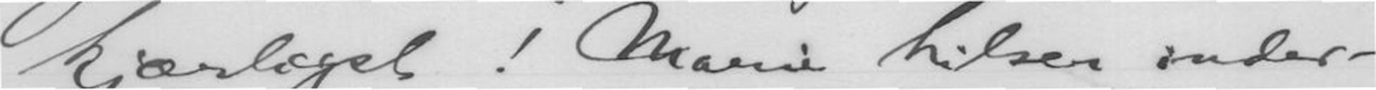kjærligst! Marie hilser inder-
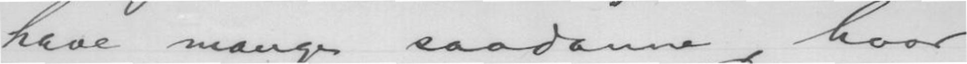have mange saadanne, hvor
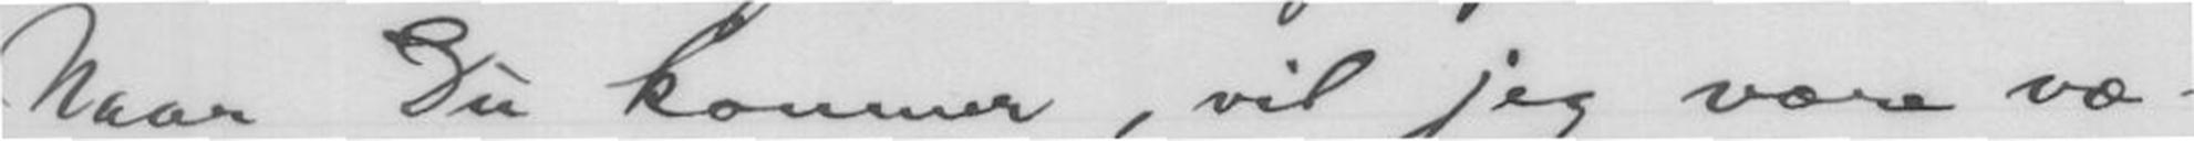Naar Du kommer, vil jeg være væ-
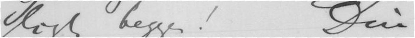ligt begge! Din
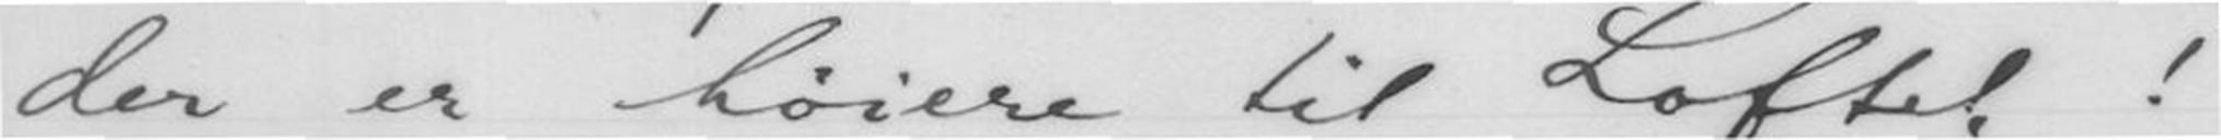der er høiere til Loftet!
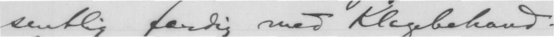sentlig færdig med Klagebehand-
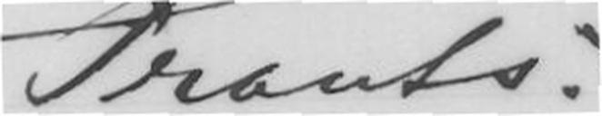Frants.
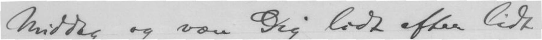Middag og væn Dig lidt efter lidt
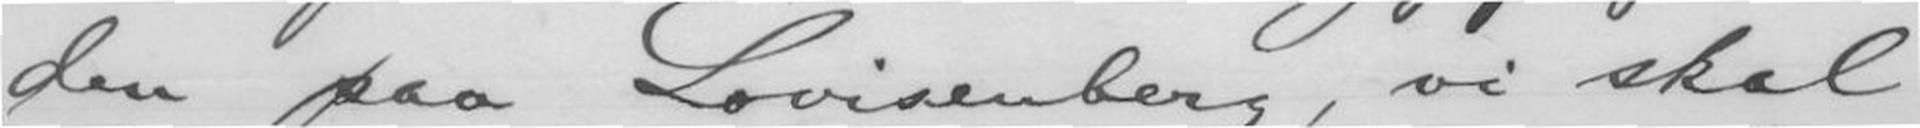den paa Lovisenberg, vi skal
